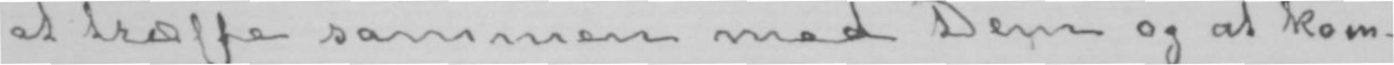at træffe sammen med Dem og at kom-
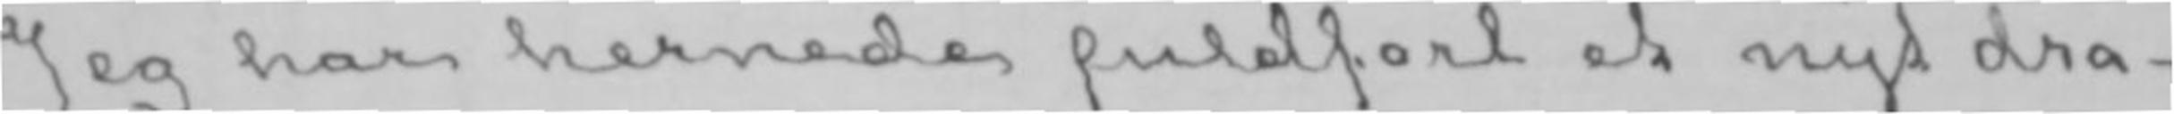Jeg har hernede fuldført et nyt dra-
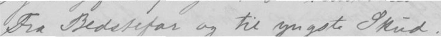Fra Bedstefar og til yngste Skud;
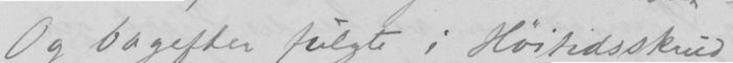Og bagefter fulgte i Høitidsskurd
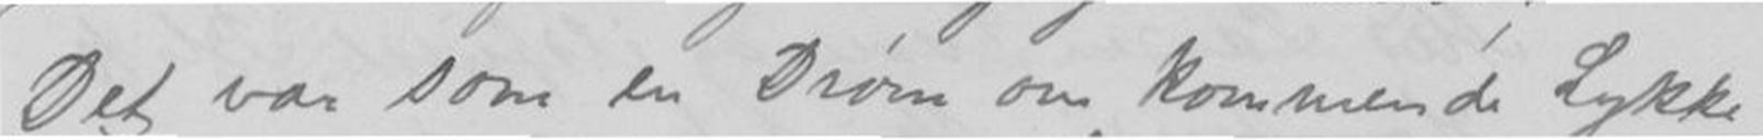Det var som en Drøm om kommende Lykke,
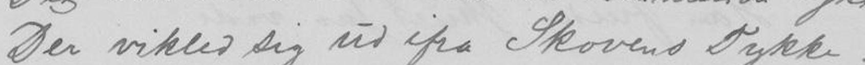Der vikled sig ud ifra Skovens Tykke -
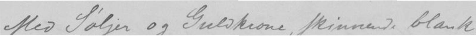Med Søljer og Guldkroner, skinnende blank,
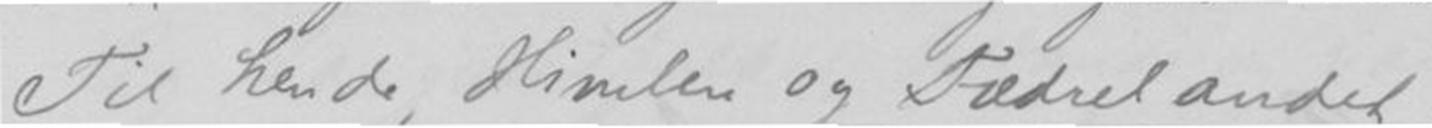Til hende, Himlen og Fædrelandet -
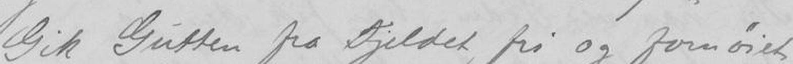Gik Gutten fra Fjeldet, fri og fornøiet,
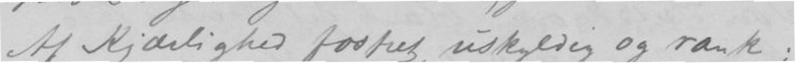Af Kjærlighed fostret, uskyldig og rank;
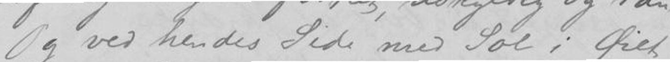Og ved hendes Side med Sol i Øiet
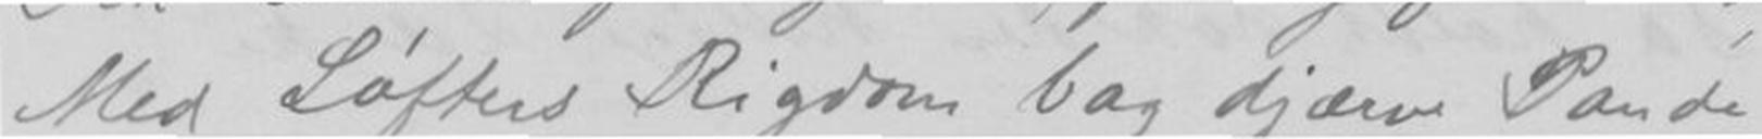Med Løfters Rigdom bag djærve Pande
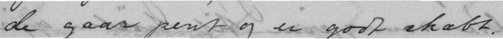de gaar pent og er godt skabt.
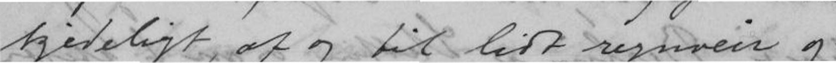kjedeligt, af og til lidt regnveir og
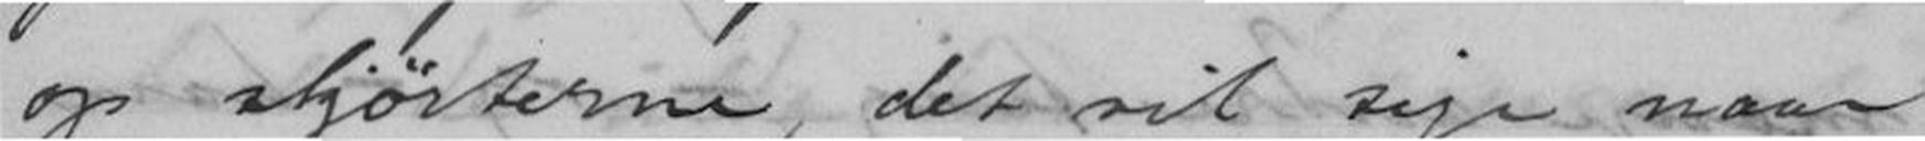op skjørterne, det vil sige naar
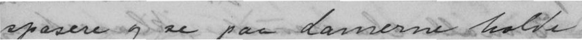spasere og se paa damerne holde
